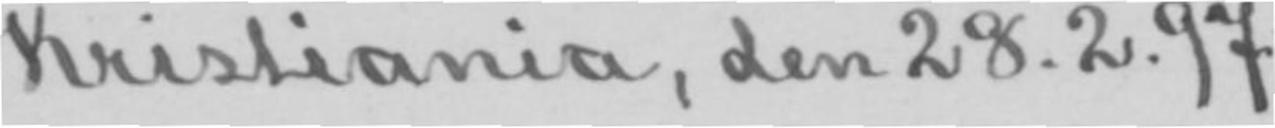Kristiania, den 28.2.97.
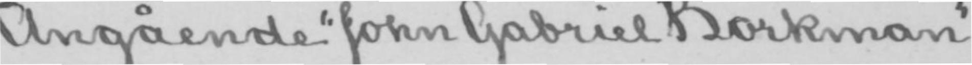Angående «John Gabriel Borkman»
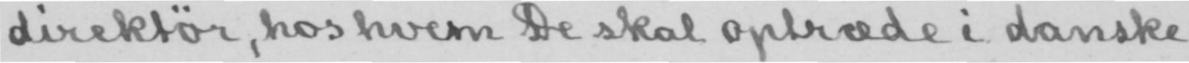direktør, hos hvem De skal optræde i danske
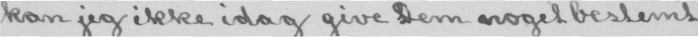kan jeg ikke idag give Dem noget bestemt
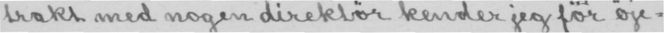trakt med nogen direktør kender jeg føor øje-
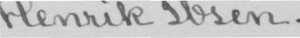Henrik Ibsen.
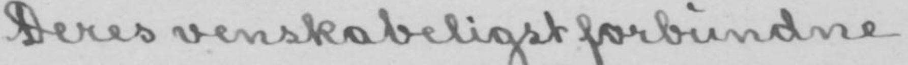Deres venskabeligst forbundne
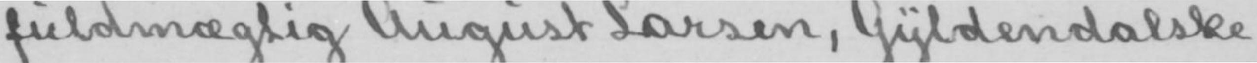fuldmægtig August Larsen, Gyldendalske
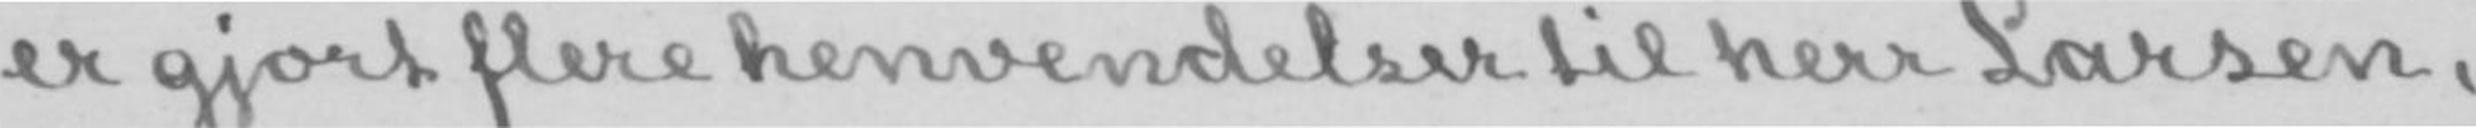er gjort flere henvendelser til herr Larsen,
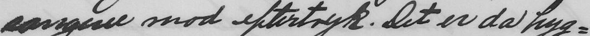sangene mod eftertryk. Det er da hyg=
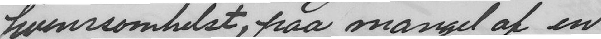hvemsomhelst, paa mangel af en
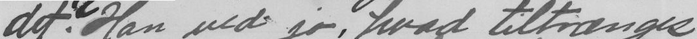det? Han ved jo, hvad tiltrænges
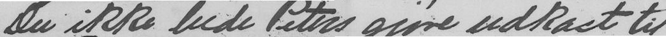Du ikke bede Peters gjøre udkast til
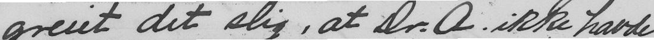greiet det slig, at Dr. A. ikke havde
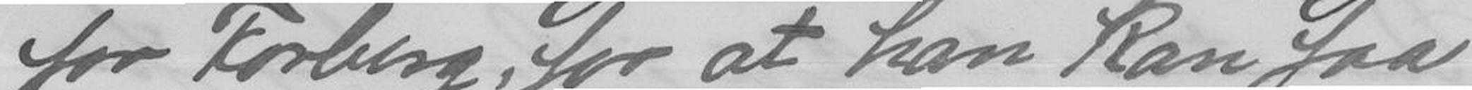for Forberg, for at han kan faa
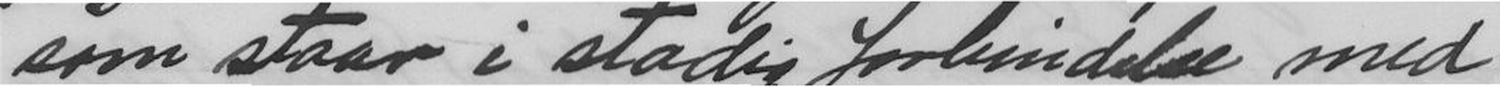som staar i stadig forbindelse med
